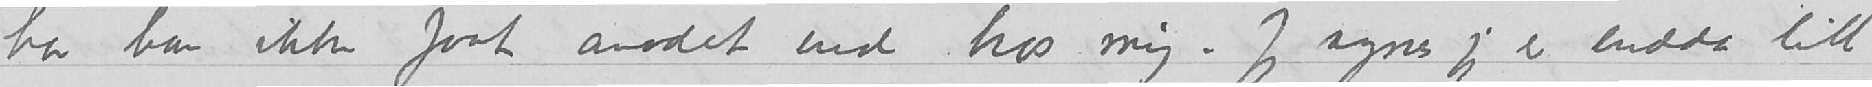har han ikke faat andet end hos mig. Jeg syns jeg er endda litt
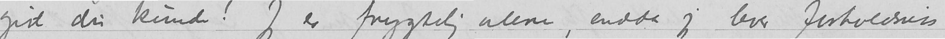gid du kunde! Jeg er frygtelig alene, endda jeg lever forholdsvis
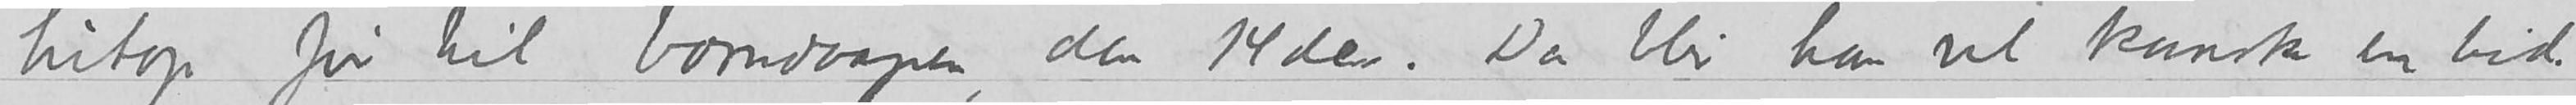hitop før til barndaapen, den 14de. Da blir han vel kanske en tid.
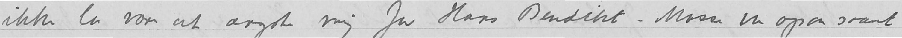ikke la være at ængste mig for Hans Bendikt – Mosse var ogsaa saant
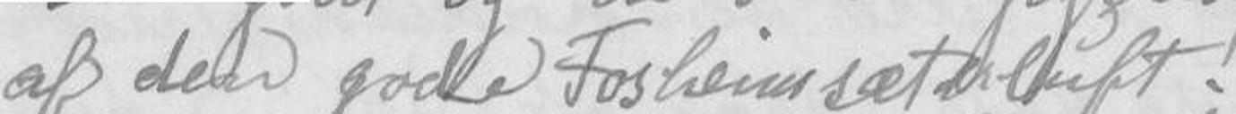af den gode Fosheimsæterluft!
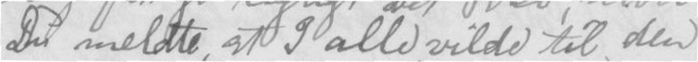Du meldte, at I alle vilde til den
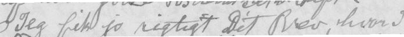Jeg fik jo rigtigt Dit Brev, hvor I
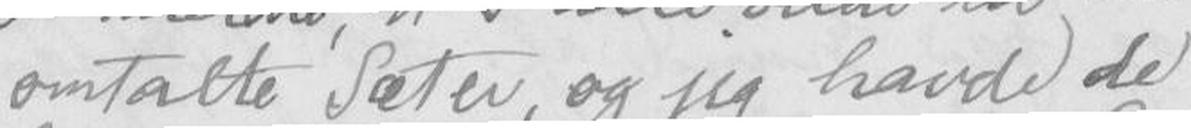omtalte Sæter, og jeg havde de
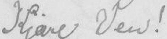Kjære Ven!
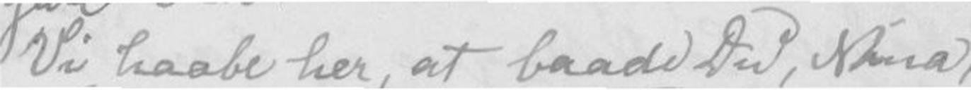Vi haabe her, at baade Du, Nina,
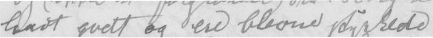havt godt og ere blivne styrkede
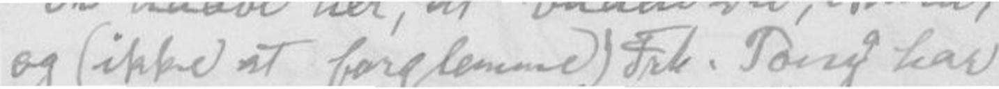og (ikke at forglemme) Frk. Tonny har
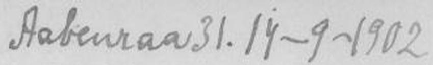Aabenraa 31. 14 - 9 - 1902
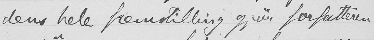dens hele fremstilling gjør forfatteren
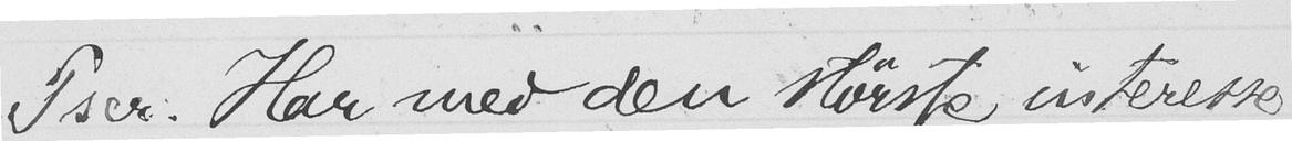P scr. Har med den største interesse
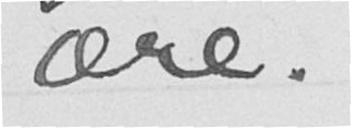ære.
